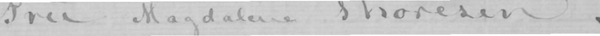Fru Magdalene Thoresen.
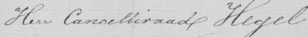Herr Cancelliraad Hegel
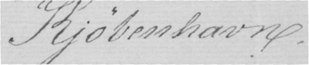Kjøbenhavn.
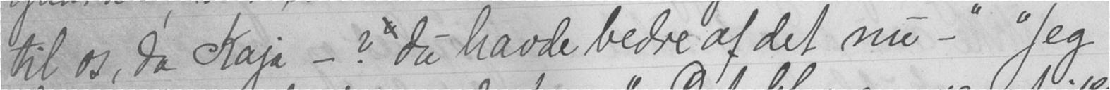til os, da Kaja -?" du havde bedre af det nu - "Jeg
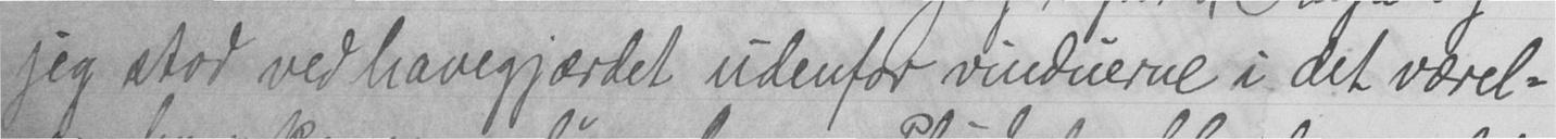jeg stod ved havegjærdet udenfor vinduerne i det værel-
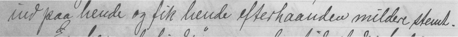ind paa hende og fik hende efterhaanden mildere stemt.
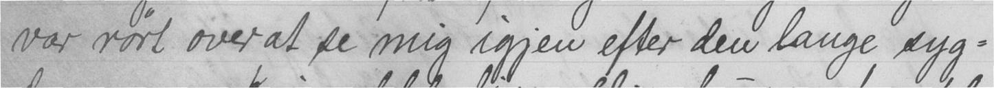var rørt over at se mig igjen efter den lange syg-
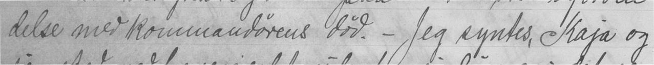delse med kommandørens død. - Jeg syntes, Kaja og
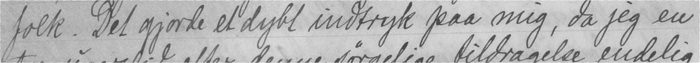folk. Det gjorde et dybt indtryk paa mig, da jeg en
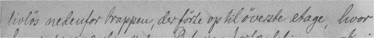livløs nedenfor trappen, der førte op til øverste etage, hvor
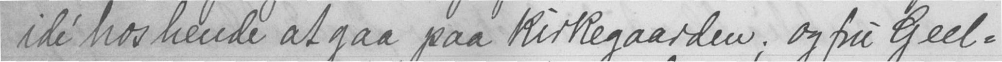idé hos hende at gaa paa kirkegaarden; og fru Geel-
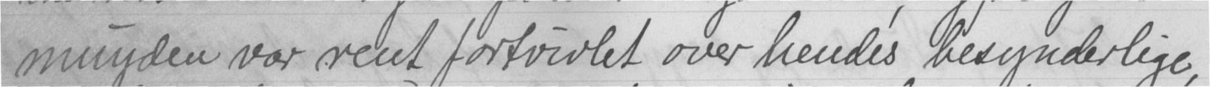muyden var rent fortvivlet over hendes besynderlige,
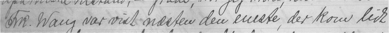Frk. Wang var vist næsten den eneste, der kom lidt
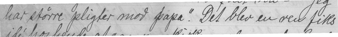har større pligter mod papa." Det blev en ren fiks
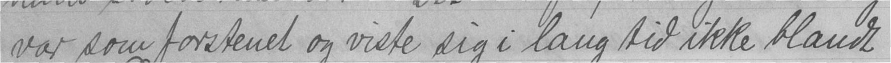var som forstenet og viste sig i lang tid ikke blandt
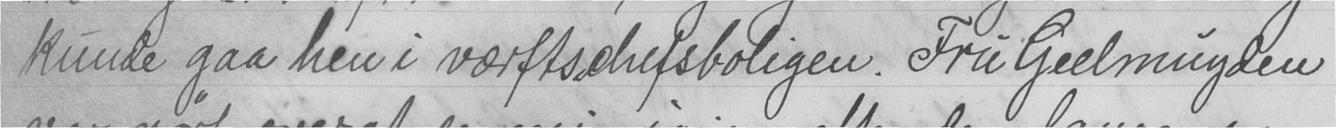kunde gaa hen i værftschefsboligen. Fru Geelmuyden
